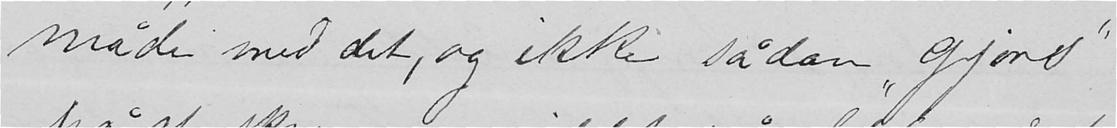måde med det, og ikke sådan "gjørs"
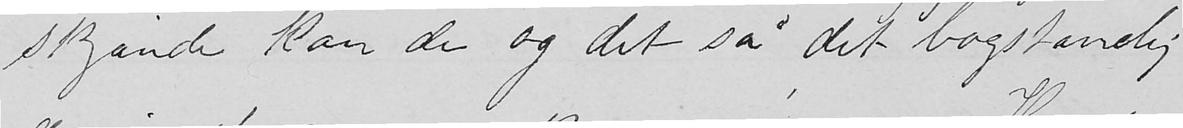skjænde kan de og det så det bogstavelig
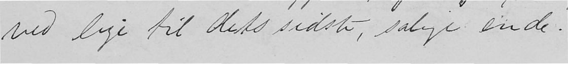ved lige til dets sidste, salige ende.
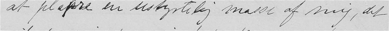at plapre en ustyrtelig masse af mig, det
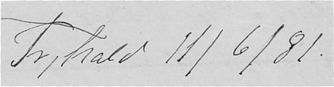Fr.hald 11/6/81.
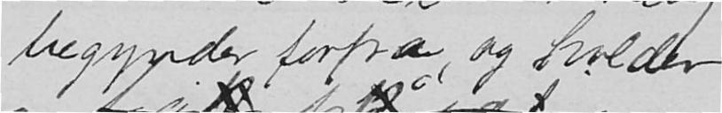begynder forfra, og holder
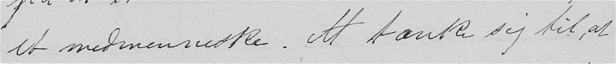et medmenneske. At tænke sig til, at
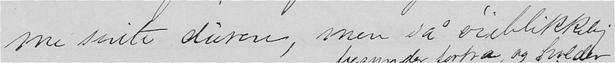me sinte duren, men så øieblikkelig
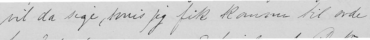vil da sige, hvis jeg fik komme til orde
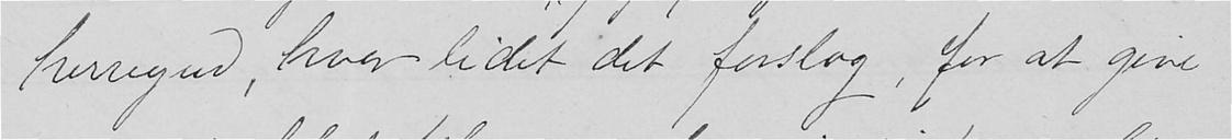herregud, hvor lidet det forslag, for at give
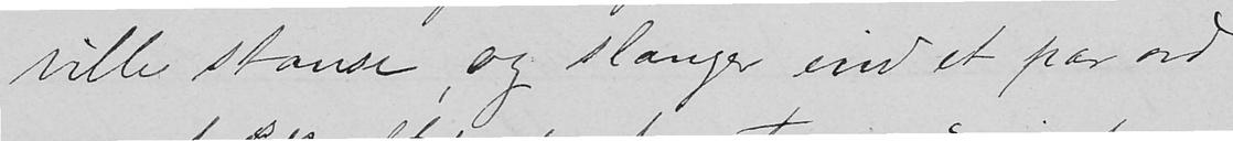ville stanse, og slænger ind et par ord
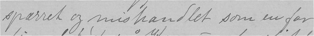spærret og mishandlet som en far-
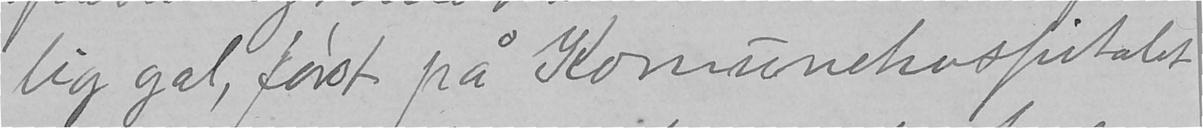lig gal, først på Komunehospitalet
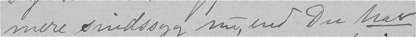mere sindssyg nu, end Du har
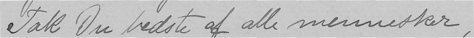Tak Du bedste af alle mennesker,
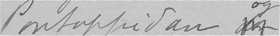Pontoppidan der
